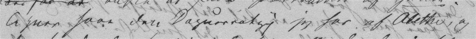Tegner saae den Daguerrotyp jeg har af Alethe, og
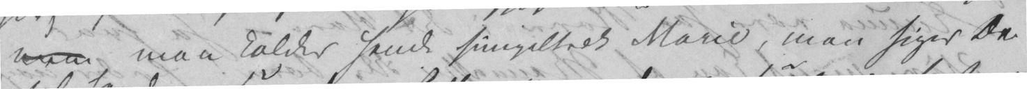men man kalder hende simpeltvæk Marie, man siger De
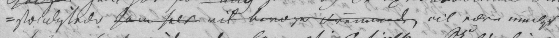-stændigheder som selv vil bevæge Fremmede, vil være mueligt
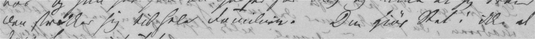den strækker sig til hele Familien. Du gjør Ret i ikke at
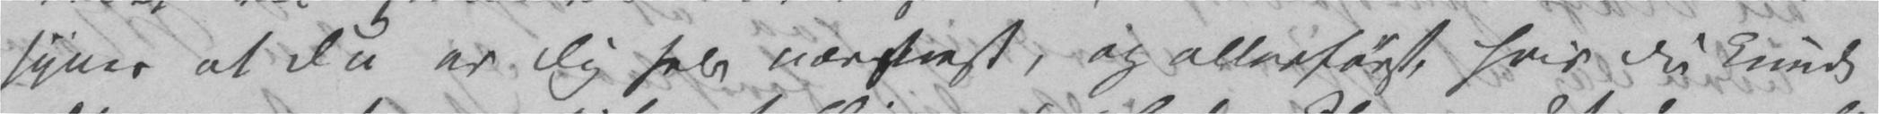synes at Du er Dig selv nærmest, og allerførst, hvis Du kunde
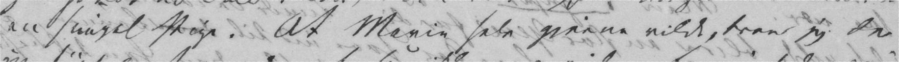en simpel Pige. At Marie selv gjerne vilde, troer jeg da
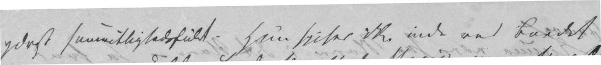yderst samvittighedsfuldt. Hun spiser ikke inde ved Bordet
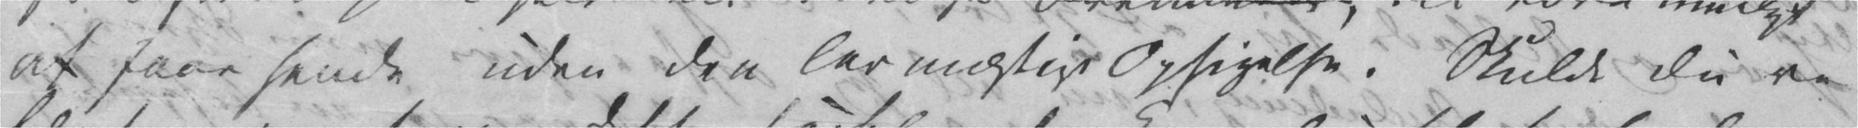at faae hende uden den lovmæssige Opsigelse. Skulde Du re
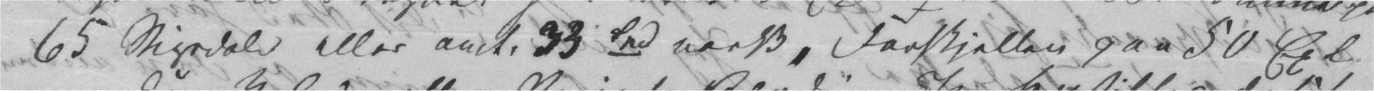65 Rigsdaler eller omt. 33 Spd norsk, Forskjellen paa 50 Exl
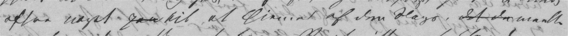afsee noget paa til et Øiemed af den Slags, det da maatte
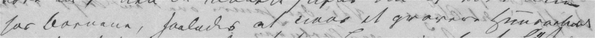hos Børnene, saaledes at naar et grovere Huusarbeide
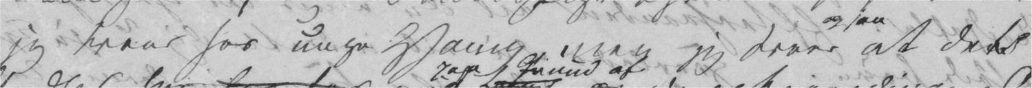jeg troer hos unge Young, men jeg troer ogsaa at det
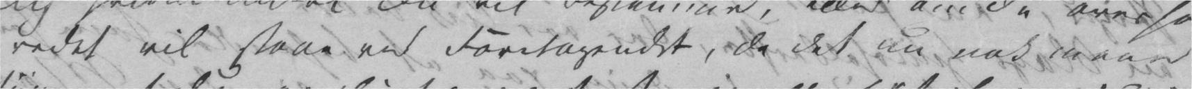vedet vil staae ved Foretagendet, da det nu nok maae
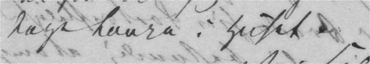tage Laura i Huset.
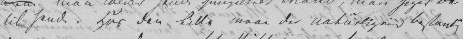til hende. Hos den Lille maae der naturligviis bestandig
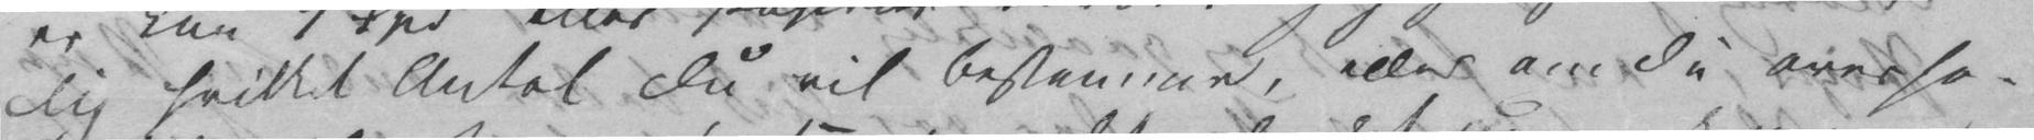Dig hvilket Antal Du vil bestemme, eller om Du overho-
